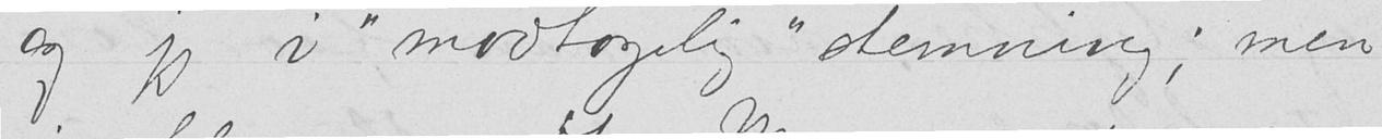og jeg i "modtagelig" stemning; men
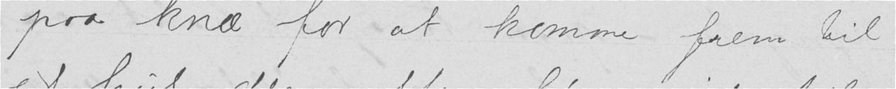paa knæ for at komme frem til
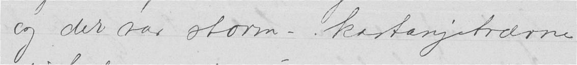og der var storm - kastanjetrærne
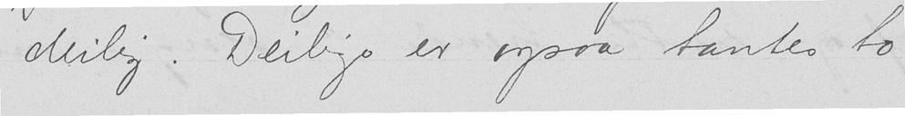deilig. Deilige er ogsaa tantes to
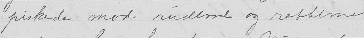piskede mod ruderne og rotterne
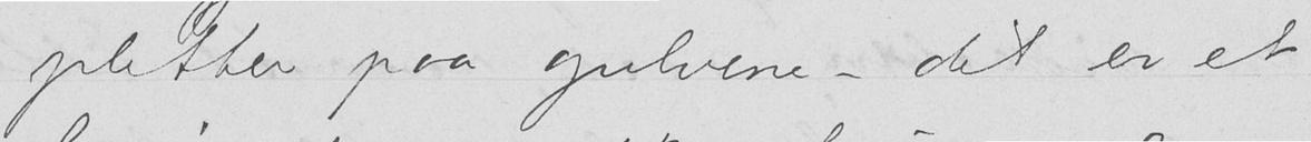pletter paa gulvene - det er et
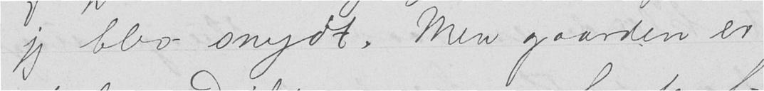jeg blev snydt. Men gaarden er
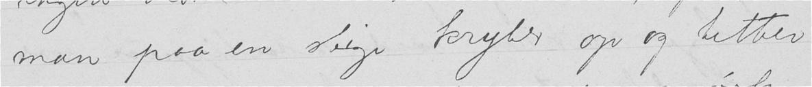man paa en stige kryber op og titter
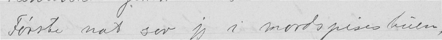Første nat sov jeg i mordspisestuen,
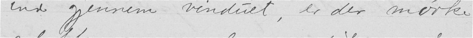ind gjennem vinduet, er der mørke
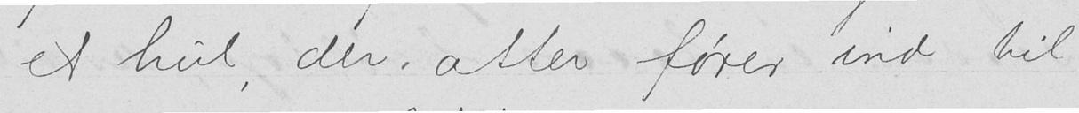et hul, der atter fører ind til
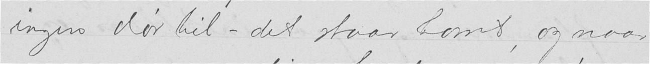ingen dør til – det staar tomt, og naar
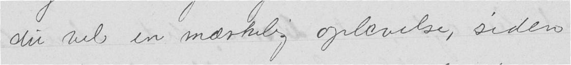du vel en mærkelig oplevelse, siden
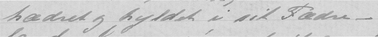hædret og hyldet i sit Fædre-
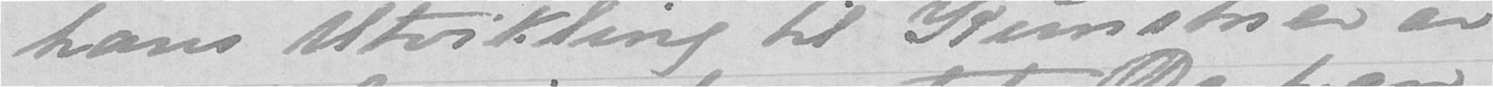hans Utvikling til Kunstner er
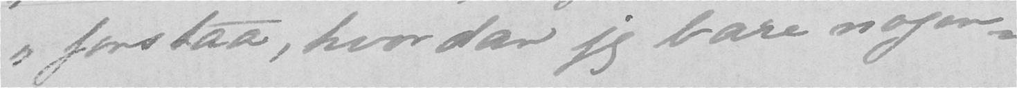" forstaa, hvor dan jeg bare nogen-
